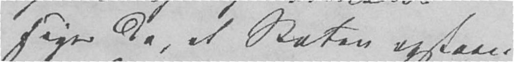siger da, at Staten opstaaer
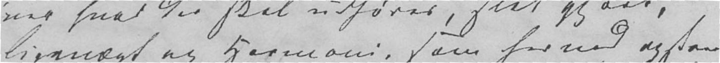Ligevægt og Harmoni, som herved opstaaer
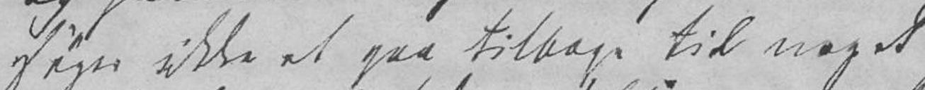søger ikke at gaa tilbage til noget
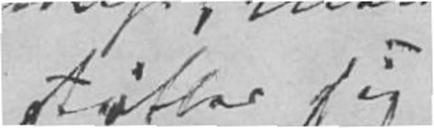støtter sig
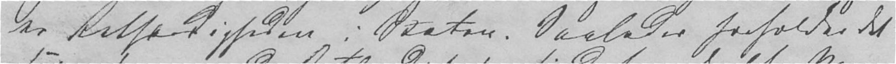er Retfærdigheden i Staten. Saaledes forholder det
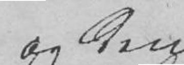og den
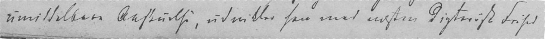umiddelbare Anskuelse, udvikler han med næsten digterisk Frihed
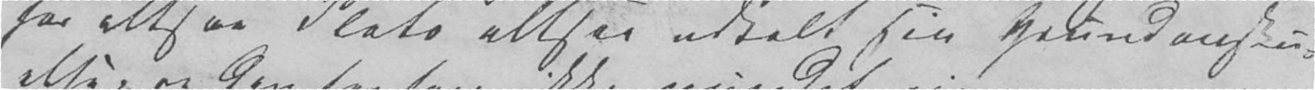har altsaa Plato altsaa udkalt sin Henndansku-
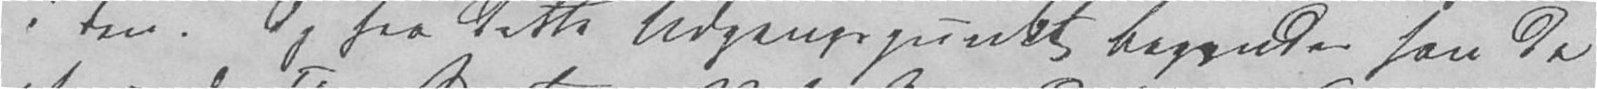i den. Og fra dette Udgangspunkt begynder han da
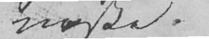neske.
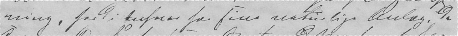ning, fordi Enhver har sine naturlige Anlæg. De
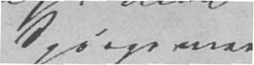Spørgsmaa-
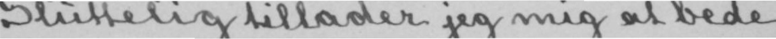Sluttelig tillader jeg mig at bede
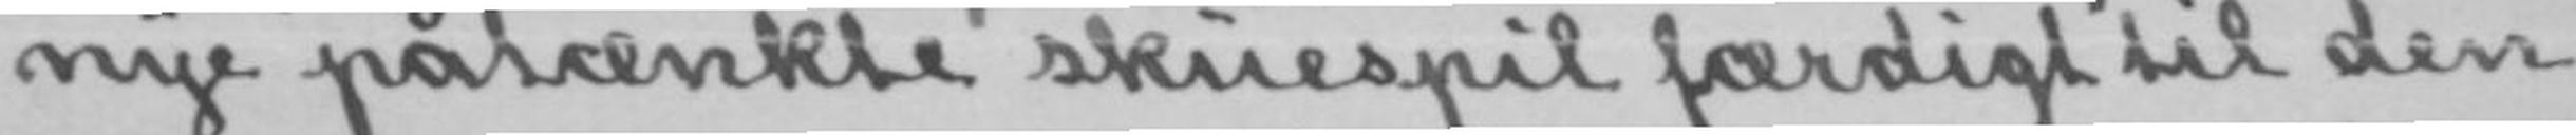nye påtænkte skuespil færdigt til den
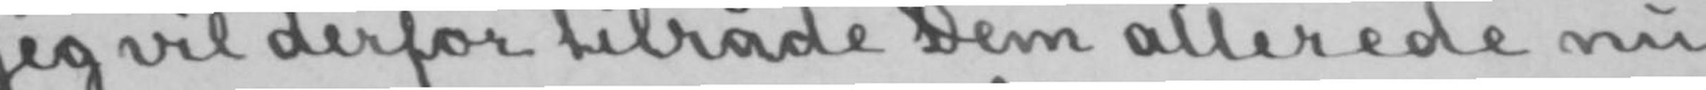Jeg vil derfor tilråde Dem allerede nu
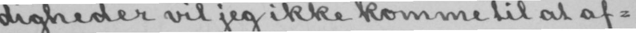digheder vil jeg ikke komme til at af-
